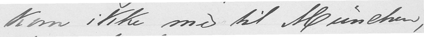kom ikke med til München
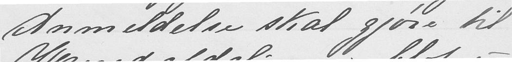Anmeldelse skal gjøre til
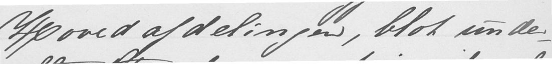Hovedafdelingen, blot under-
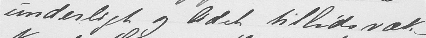underligt og lidet tillidsvæk-
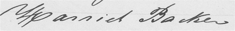Harriet Backer
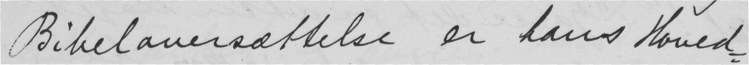Bibeloversættelse er hans Hoved-
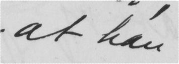at han
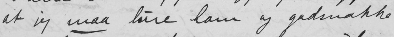at jeg maa lure ham og godsnakke
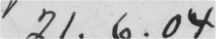21.6.04
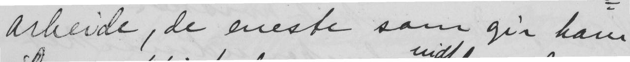arbeide, de eneste som gir ham
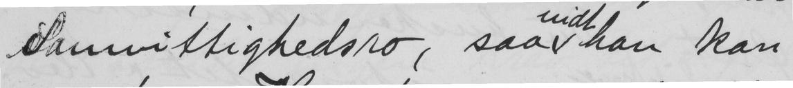han kan
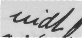vidt.
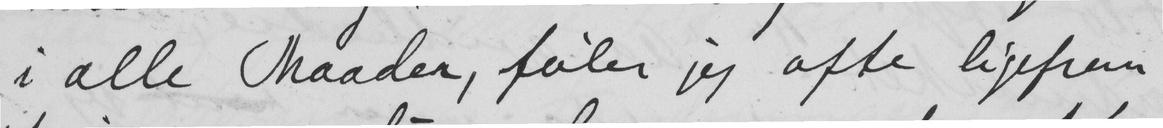i alle Maader, føler jeg ofte ligefrem
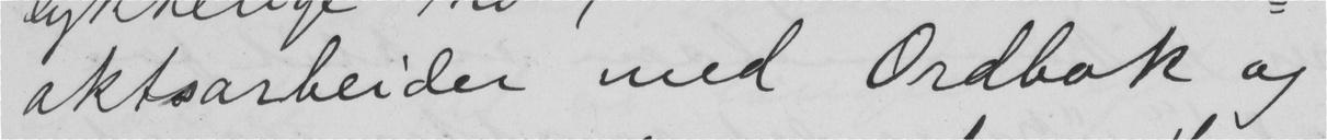aktsarbeider med Ordbok og
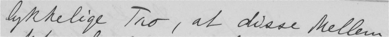lykkelige Tro, at disse Mellem
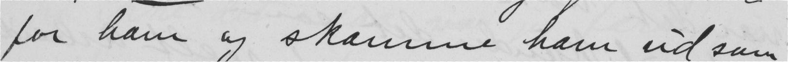for ham og skamme ham ud som
